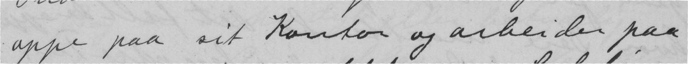oppe paa sit Kontor og arbeider paa
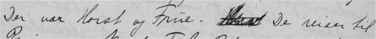Der var Horst og Frue.  De reiser til
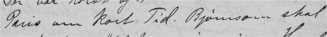Paris om kort Tid. Bjørnson skal
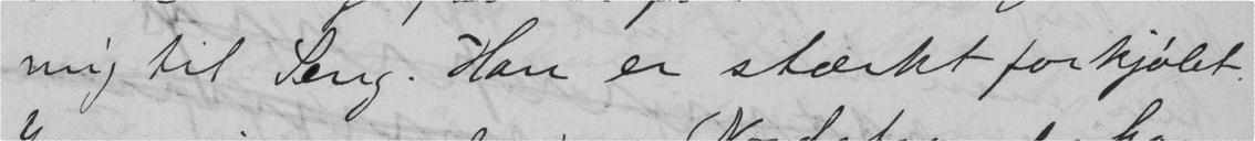mig til Seng. Han er stærkt forkjølet.
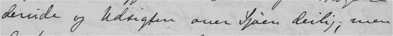derude og Udsigten over Sjøen deilig; men
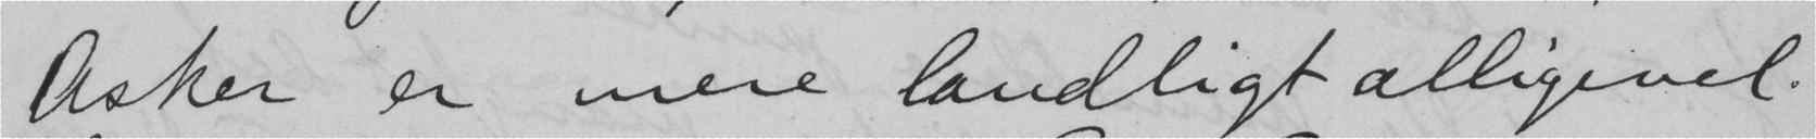Asker er mere landligt alligevel.
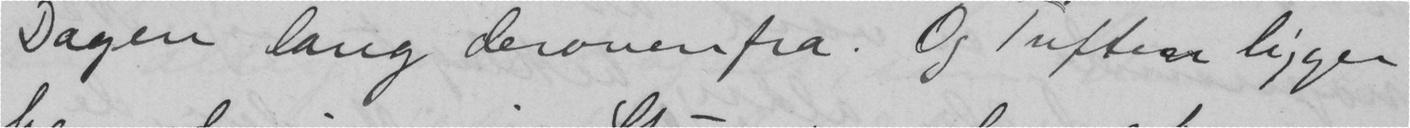Dagen lang derovenfra. Og Tuften ligger
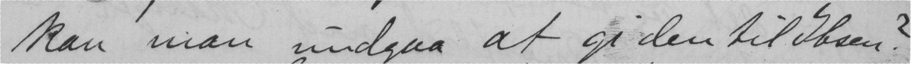kan man undgaa at gi den til Ibsen?
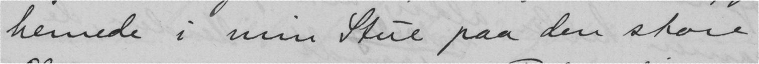hernede i min Stue paa den store
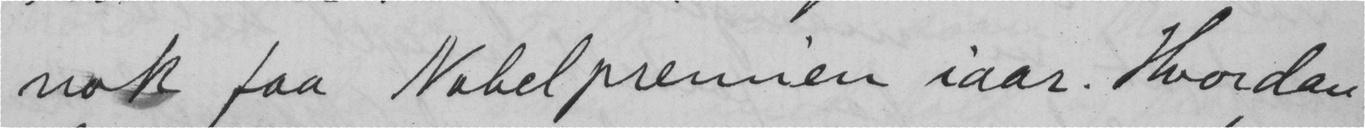nok faa Nobelprennen iaar. Hvordan
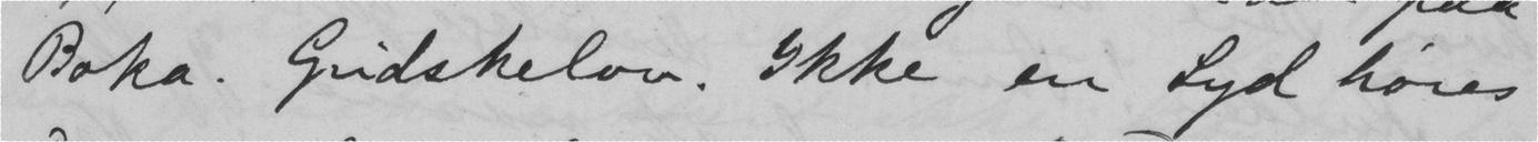Boka. Grdskelov. Ikke en Lyd høres
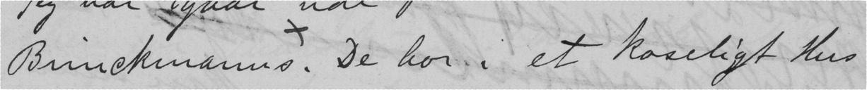Brinckmanns. De bor i et koseligt Hus
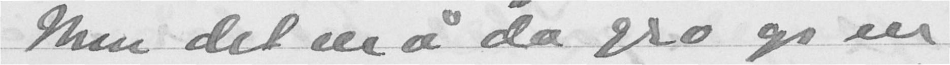Men det må da gro op en
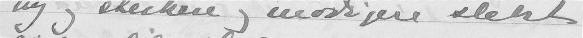ny og sterkere og modigere slekt
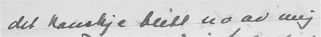det kanskje blitt no av mig.
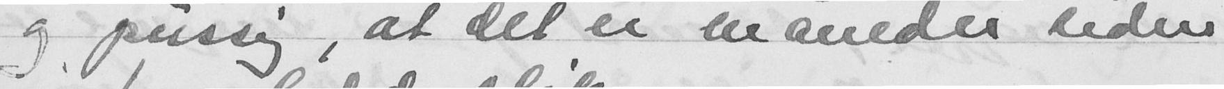og pussig, at det er måneder siden
